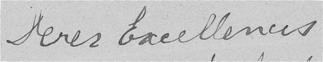Deres Excellences
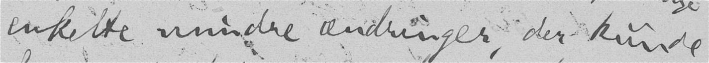enkelte mindre ændringer, der kunde
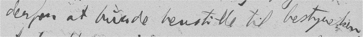derfor at burde henstille til bestyrelsen,
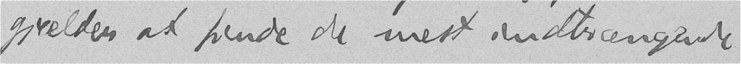gjælder at finde de mest indtrængende
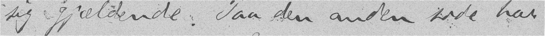sig gjældende. Paa den anden side har
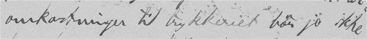omkostninger til trykkeriet bør jo ikke
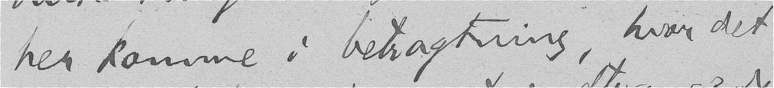her komme i betragtning, hvor det
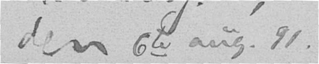den 6te aug. 91.
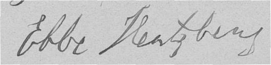Ebbe Hertzberg.
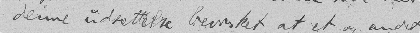denne udsættelse bevirket, at et og andet
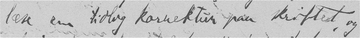læse en tidlig korrektur paa skriftet, og
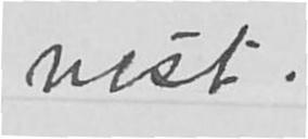visst.
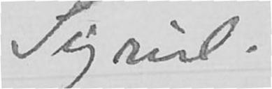Sigrid.
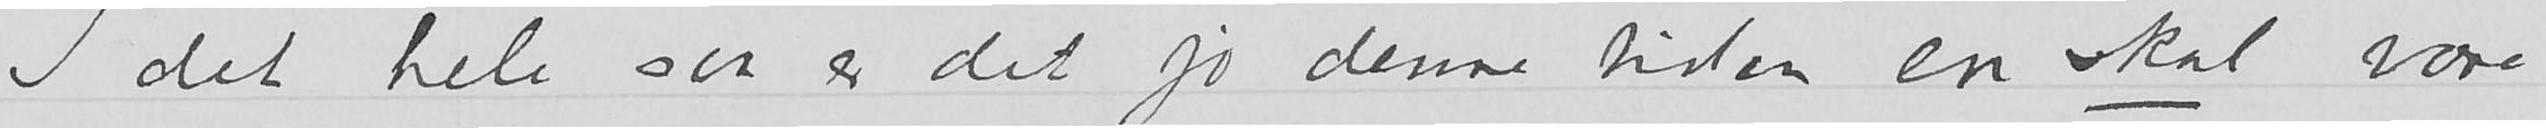I det hele saa er det jo denne tiden en skal være
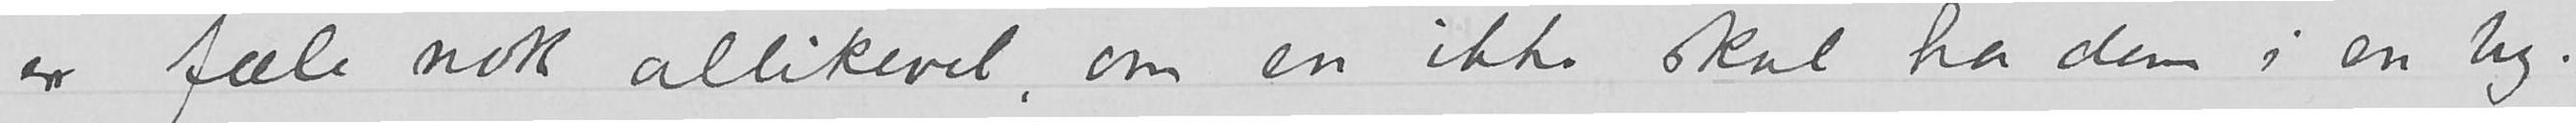er fæle nok allikevel, om en ikke skal ha dem i en by.
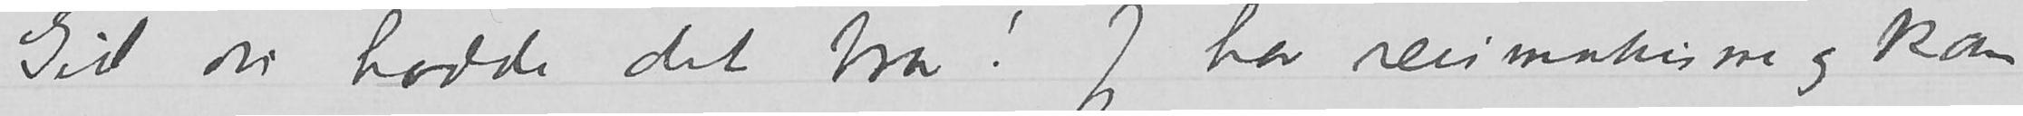Gid du hadde det bra! Jeg har reumatisme og kan
